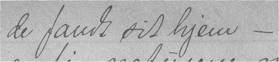de fandt sit hjem -
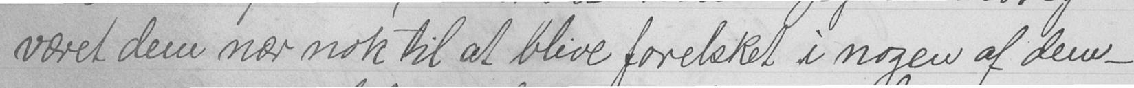været dem nær nok til at blive forelsket i nogen af dem -
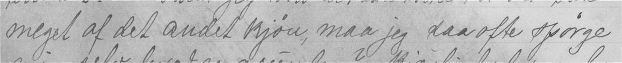meget af det andet kjøn, maa jeg saa ofte spørge
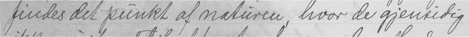findes det punkt af naturen, hvor de gjensidig
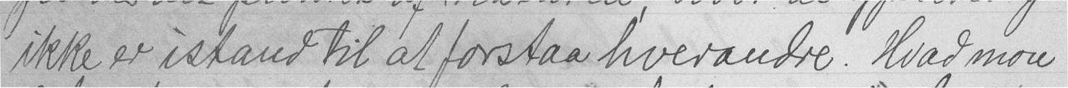ikke er istand til at forstaa hverandre. Hvad mon
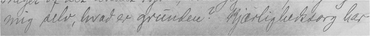mig selv, hvad er grunden. Kjærlighedssorg har
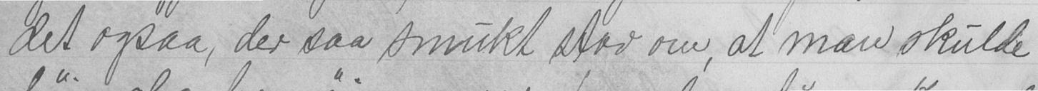det ogsaa, der saa smukt stod om, at man skulde
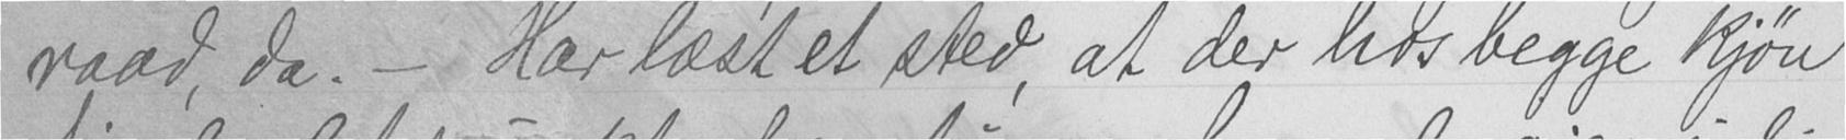raad, da. - Har læst et sted, at der hos begge kjøn
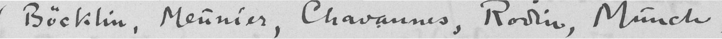(Bøcklin, Meunier, Chavannes, Rodin, Munch,
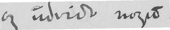og udvide noget.
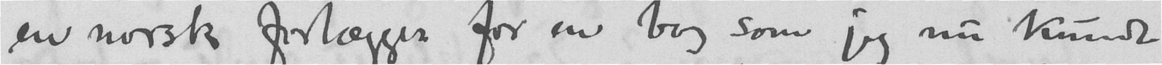en norsk forlægger for en bog som jeg nu kunde
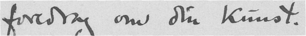foredrag om din kunst.
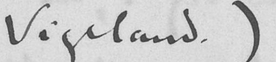Vigeland).
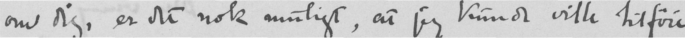om dig, er det nok muligt, at  jeg kunde ville tilføie
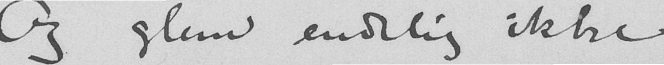Og glem endelig ikke
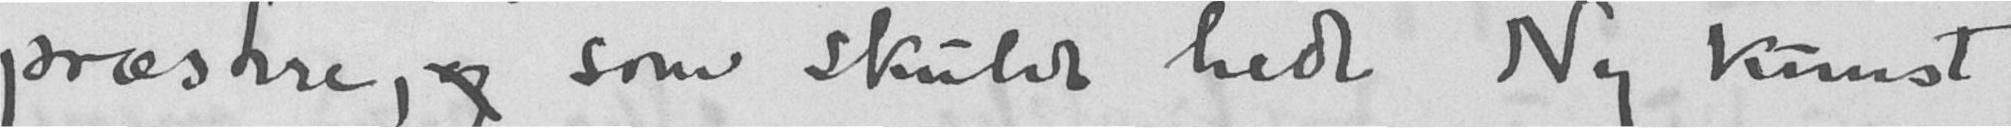præstere, og som skulde hede "Ny kunst"
